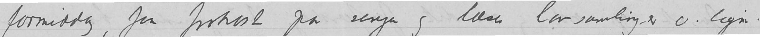formiddag, faar frokost paa sengen og læser lovsamlinger o.lign
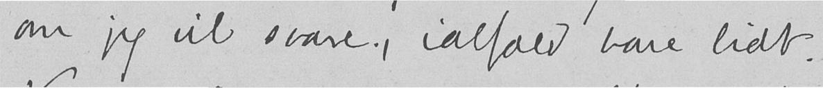om jeg vil svare, ialfald bare lidt.
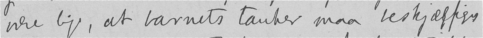være lige, at barnets tanker maa beskjæftiges
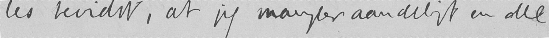les bevidst, at jeg mangler aandeligt en del
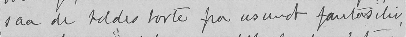saa de holdes borte fra usundt fantasiliv,
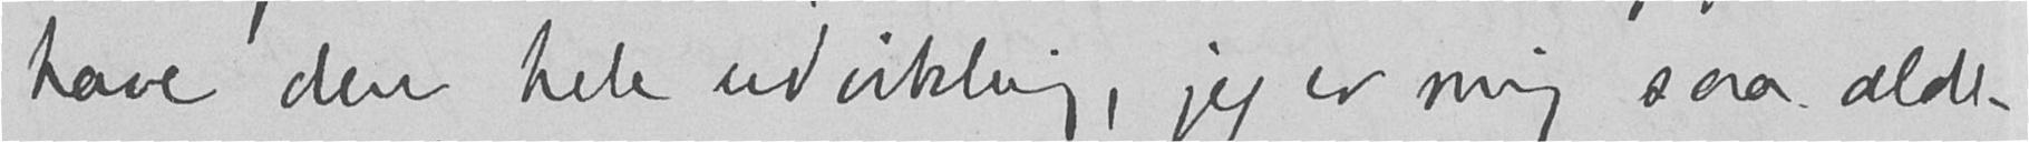have den hele udvikling, jeg er mig saa alde-
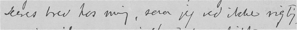Deres brev hos mig, saa jeg ved ikke rigtig,
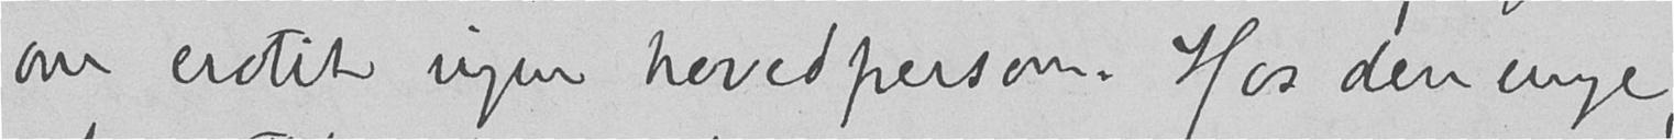om erotik ingen hovedperson. Hos den unge,
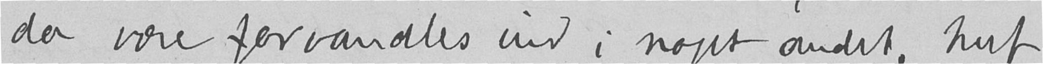da være forvandles ind i noget andet, huf
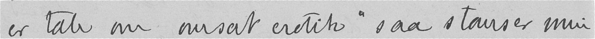er tale om "omsat erotik" saa stanser min
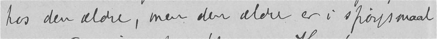hos den ældre, men den ældre er i spørgsmaal
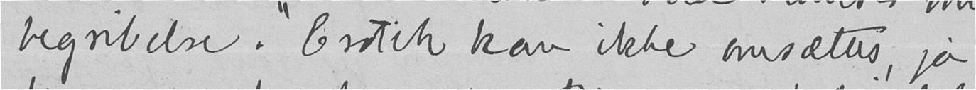begribelse. Erotik kan ikke omsættes, jo
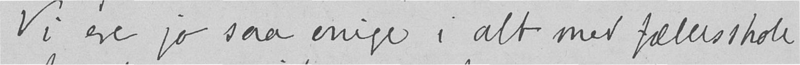Vi ere jo saa enige i alt med fællesskole
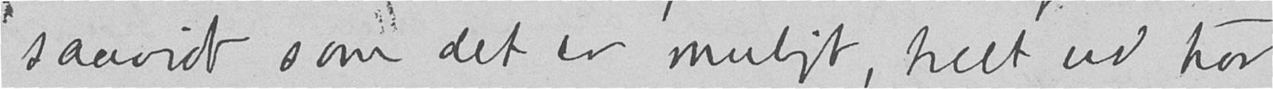saavidt som det er muligt, helt ud tror
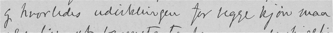og hvorledes udviklingen for begge kjøn maa
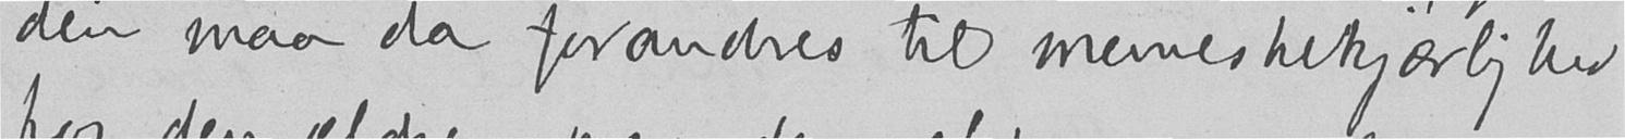den maa da forandres til menneskekjærlighed
